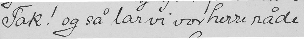Tak! og så lar vi vor herre råde
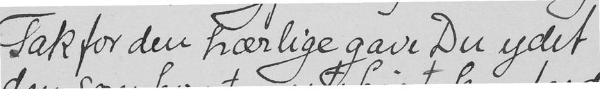Tak for den hærlige gave Du ydet
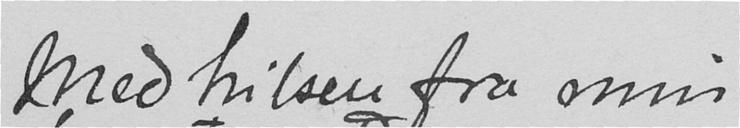Med hilsen fra min
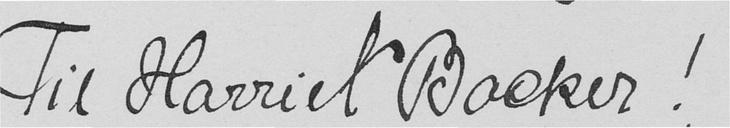Til Harriet Backer!
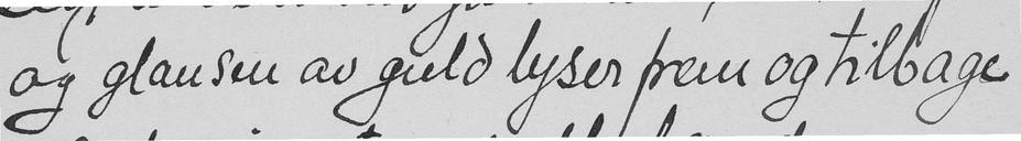og glansen av guld lyser frem og tilbage
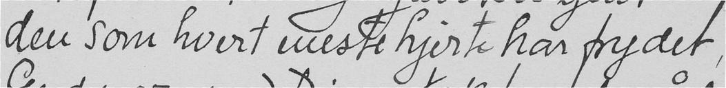den som hvert meste hjerte har frydet,
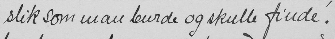slik som man burde og skulle finde.
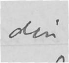din
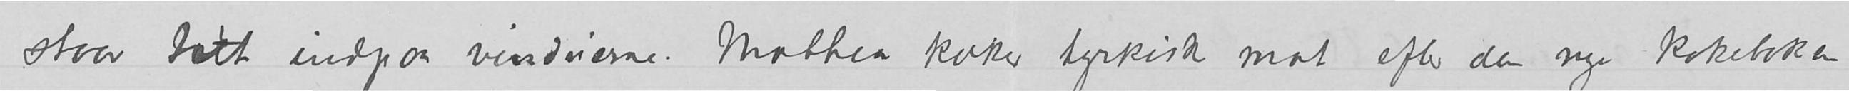staar tætt indpaa vinduerne. Matthea koker tyrkisk mat efter den nye kokeboken
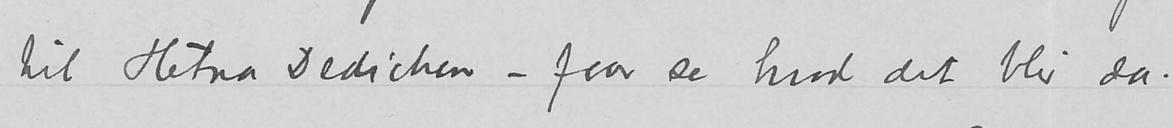til Hetna Dedichen – faar se hvad det blir da.
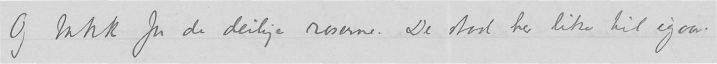Og takk for de deilige roserne. De stod her like til igaar.
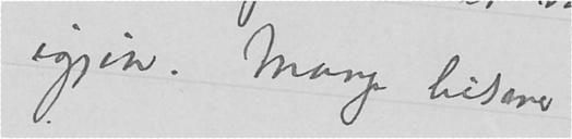igjen.  Mange hilsener
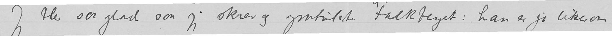Jeg blev saa glad saa jeg skrev og gratulerte Falkberget; han er jo likesom
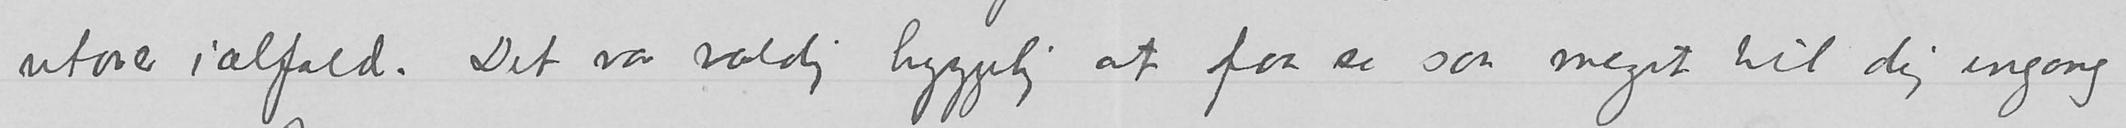utover ialfald. Det var veldig hyggelig at faa se saa meget til dig engang
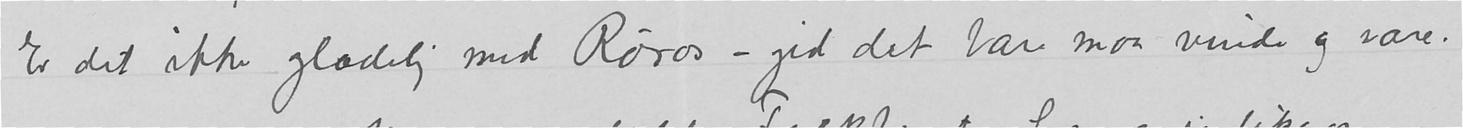Er det ikke glædelig med Røros – gid det bare maa vinde og vare.
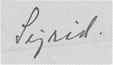Sigrid.
